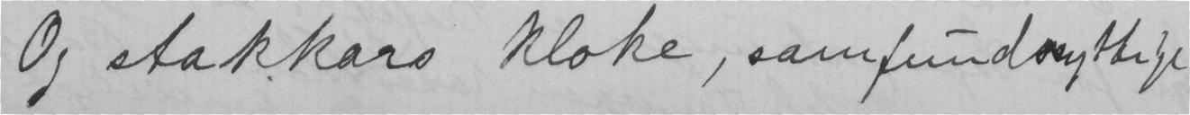Og stakkars kloke, samfundsyttige
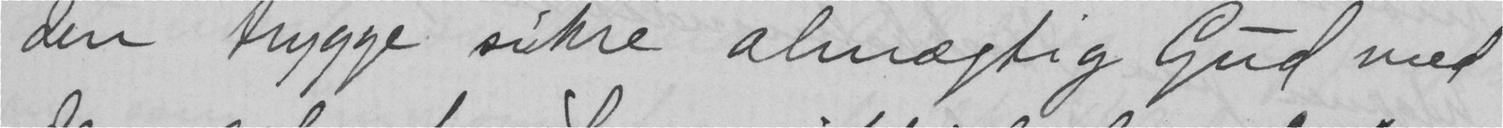den trygge sikre almægtig Gud med
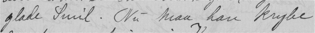glade Smil. Nu Maa han Krybe
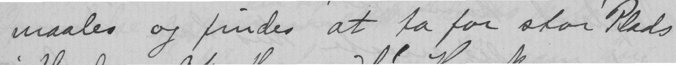maales og findes at ta for stor Plads
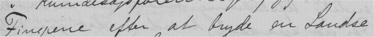Fingrene efter at bryde en Landse
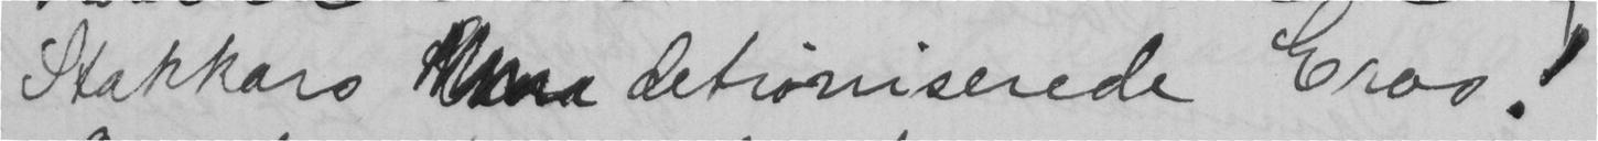Stakkars  detroniserede Eros!
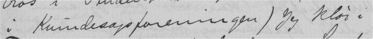i Kvindesagsforeningen) Jeg klør i
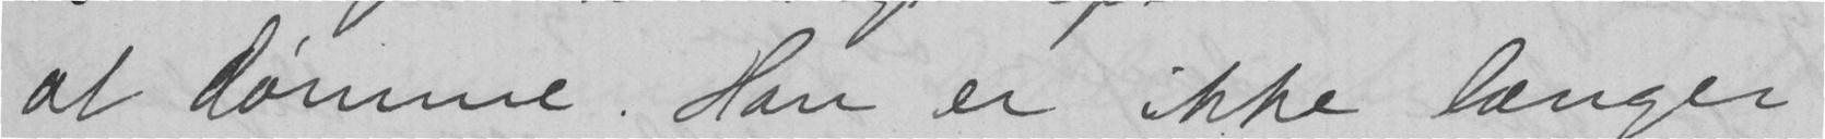at dømme. Han er ikke længer
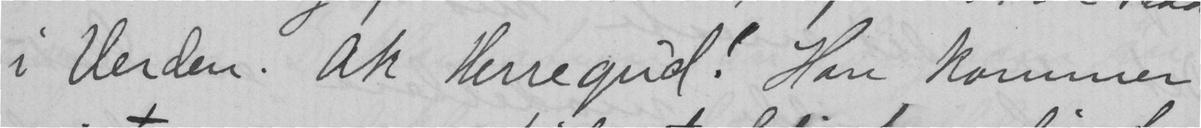i verden. Ak Herregud! Han kommer
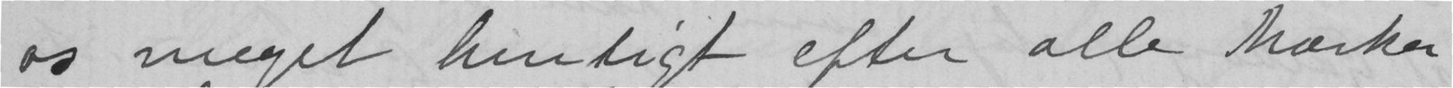os meget hurtigt efter alle Mærker
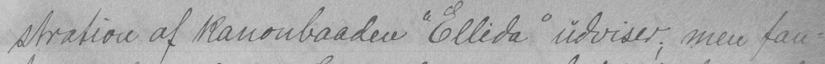stration af kanonbaaden "Ellida" udviser; men fan-
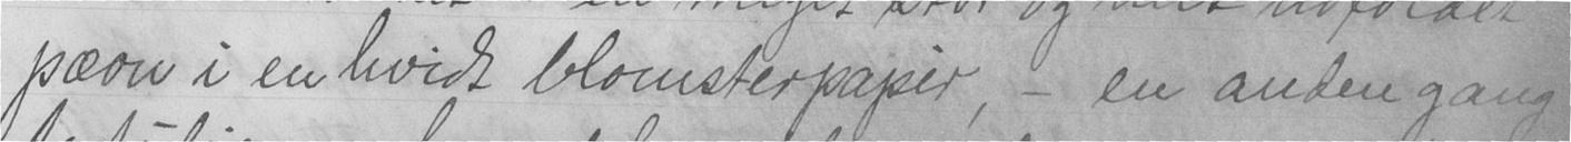pæon i en hvidt blomsterpapir, - en anden gang
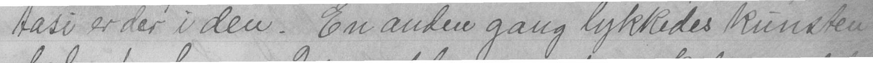tasi er der i den. En anden gang lykkedes kunsten
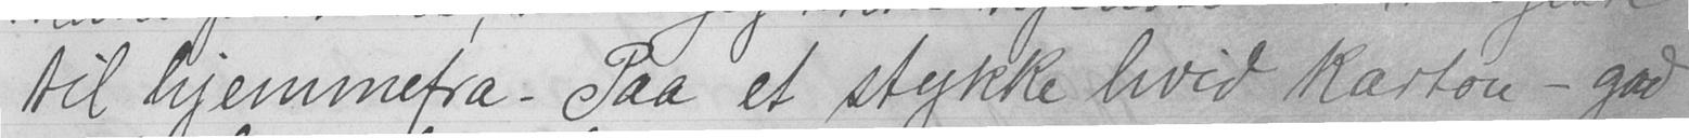til hjemmefra. Saa et stykke hvid karton - gud
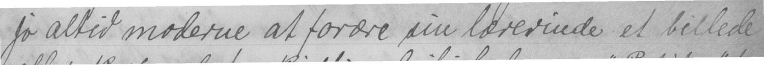jo altid moderne at forære sin lærerinde et billede
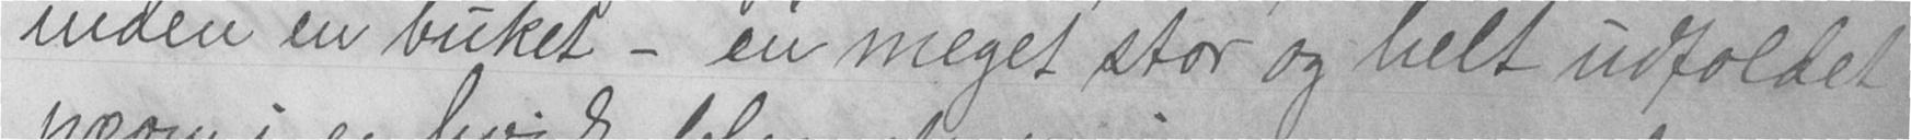inden en buket - en meget stor og helt udfoldet
